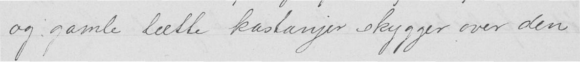og gamle tætte kastanjer skygger over den
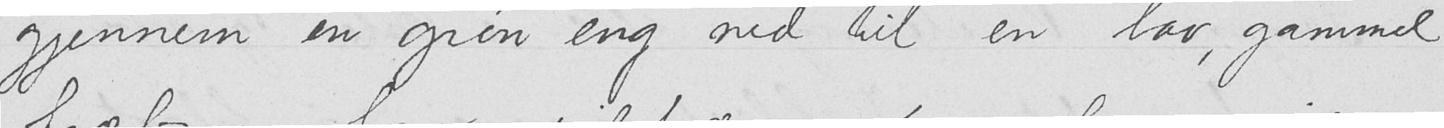gjennem en grøn eng ned til en lav, gammel
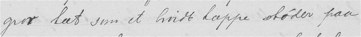gror tæt som et hvidt tæppe støder paa
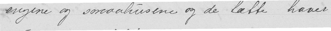engene og smaahusene og de tætte haver
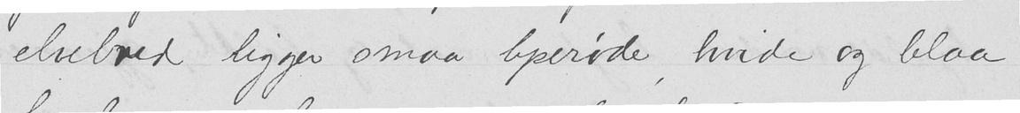elvebred ligger smaa lyserøde, hvide og blaa
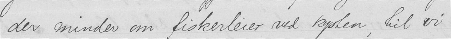der minder om fiskerleier ved kysten, til vi
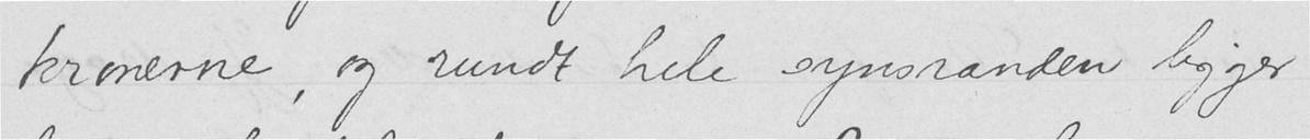kronerne, og rundt hele synsranden ligger
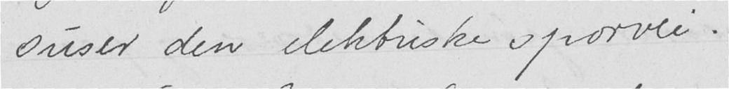suser den elektriske sporvei.
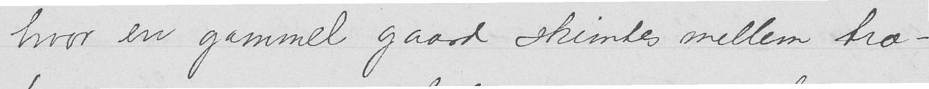hvor en gammel gaard skimtes mellem træ-
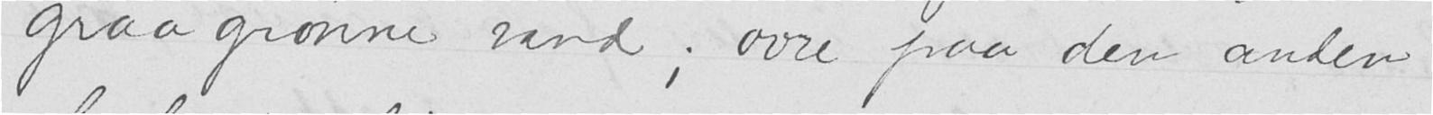graagrønne vand; ovre paa den anden
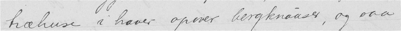træhuse i haver opover bergknauser, og saa
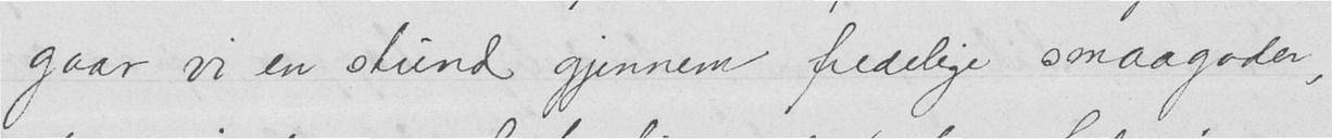gaar vi en stund gjennem fredelige smaagader,
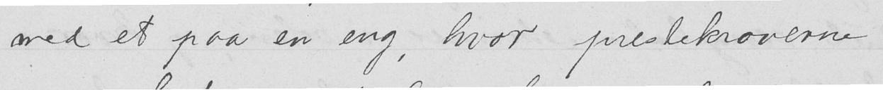med et paa en eng, hvor prestekraverne
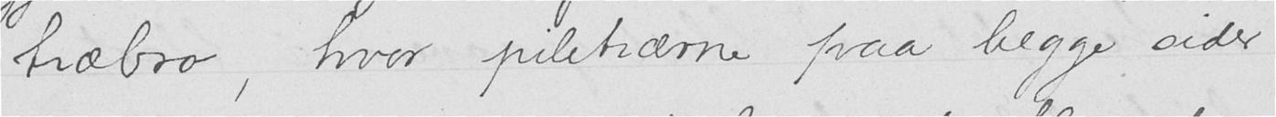træbro, hvor piletrærne paa begge sider
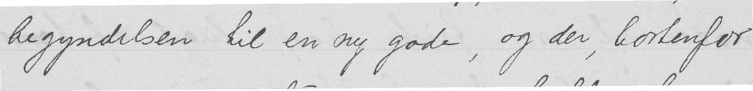begyndelsen til en ny gade, og der, bortenfor
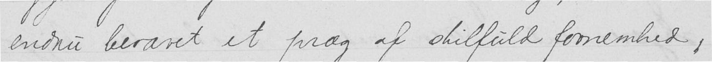endnu bevaret et præg af stilfuld fornemhed,
